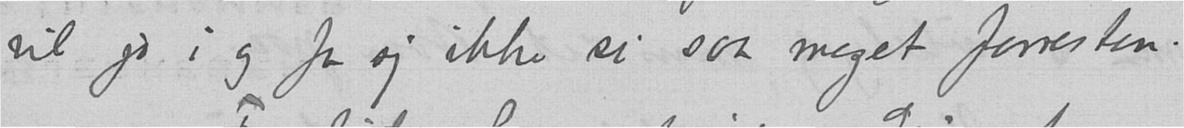vil jo i og for sig ikke si saa meget forresten.
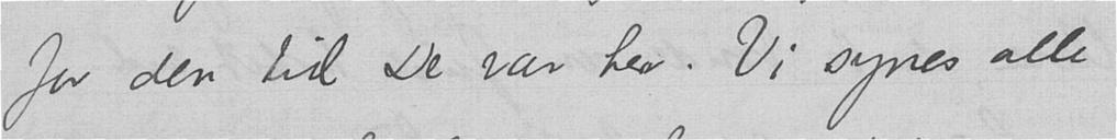for den tid De var her. Vi synes alle
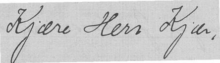Kjære Herr Kjær,
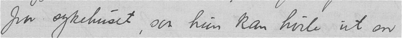fra sykehuset, saa hun kan hvile ut en
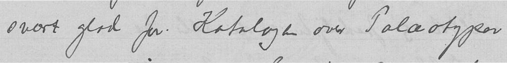svært glad for. Katalogen over Palæotyper
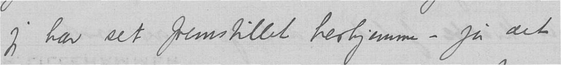jeg har set fremstillet herhjemme – ja det
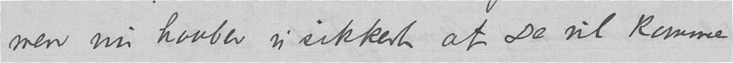men nu haaber vi sikkert at De vil komme
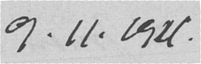9.11.1926.
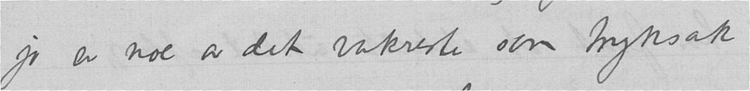jo er noe av det vakreste som tryksak
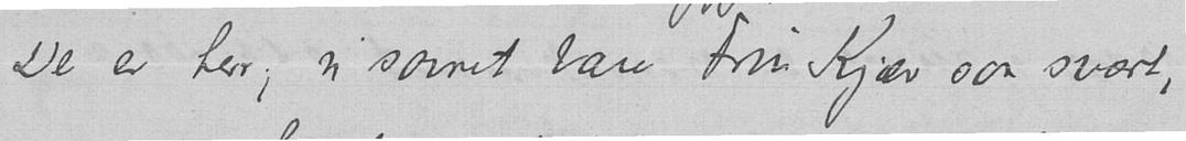De er her; vi savnet bare Fru Kjær saa svært,
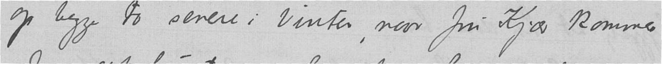op begge to senere i vinter naar fru Kjær kommer
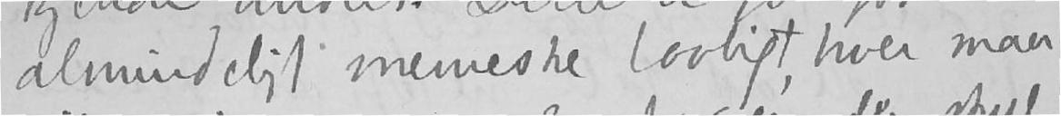almindeligt menneske lovligt, hver maa
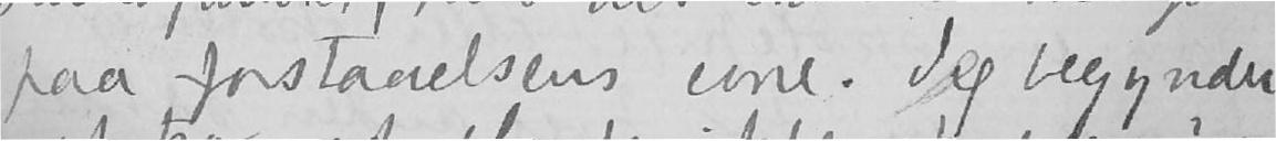paa forstaaelsens evne. Jeg begynder
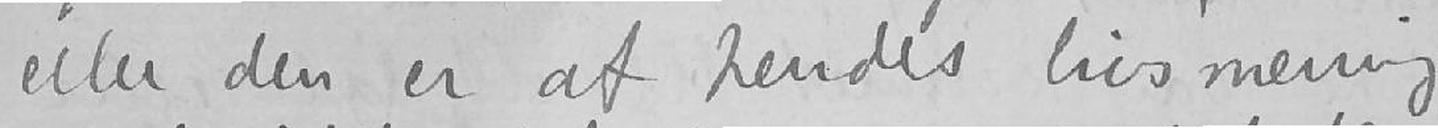eller den er af hendes livs mening
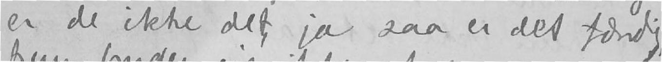er de ikke det, ja saa er det færdig,
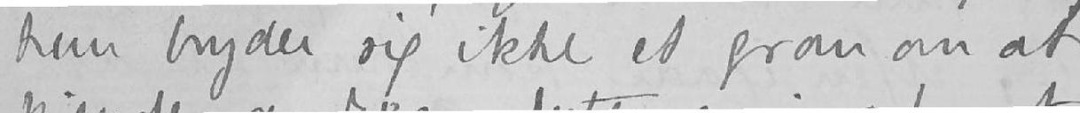hun bryder sig ikke et gram om at
